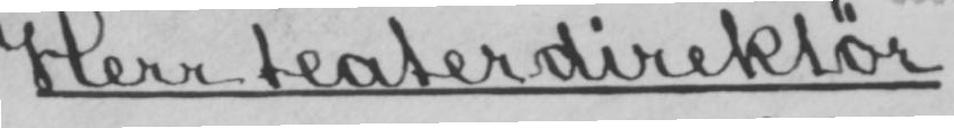Herr teaterdirektør
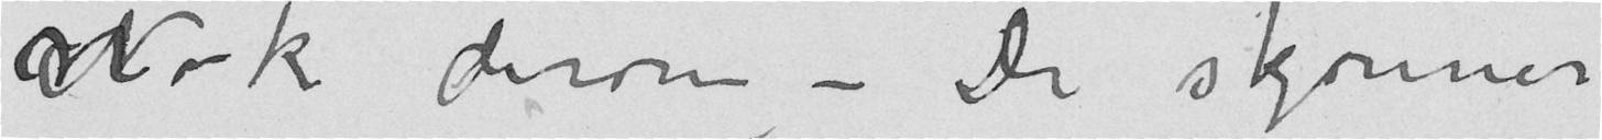nok derom – De skjønner
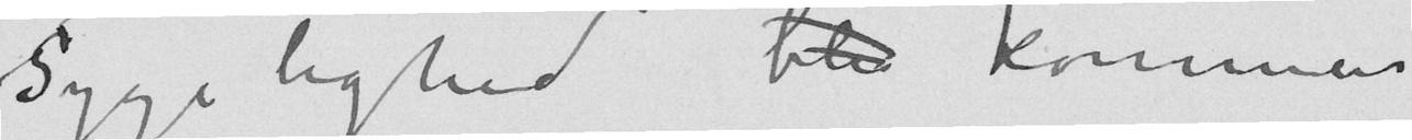Sygelighed bl kommen
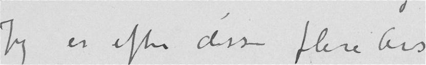Jeg er efter disse flere Års
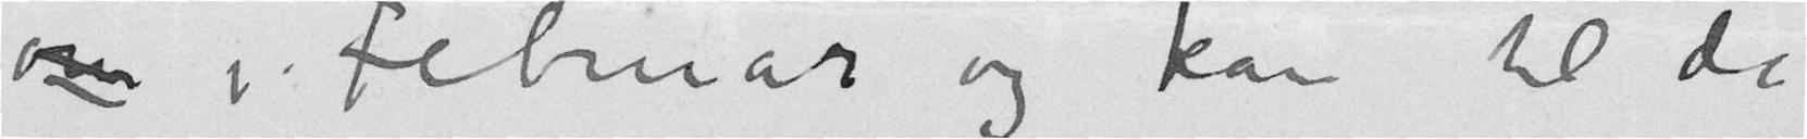om i Februar og kan til da
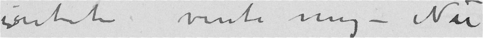intet vente mig – Nu
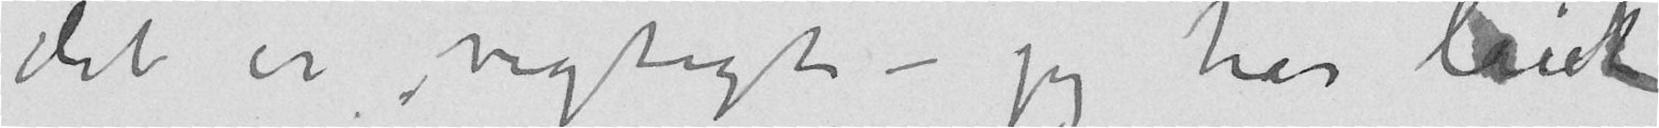det er vigtigt – jeg har leiet
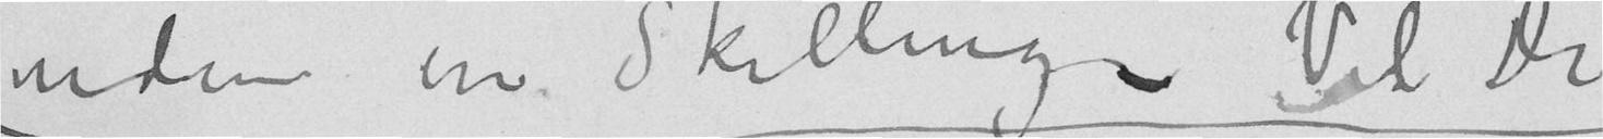uden en Skilling – Vil De
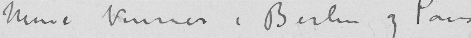Mine Venner i Berlin og Paris
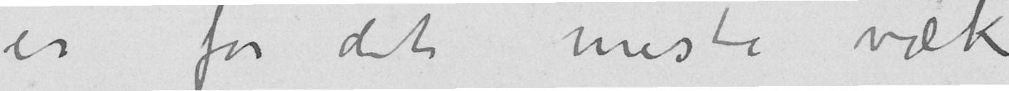er for det meste væk
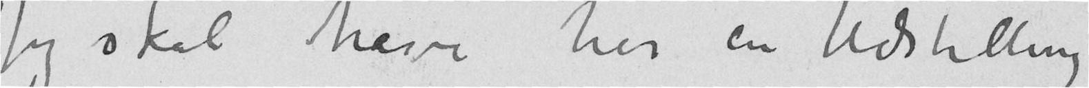Jeg skal have her en Udstilling
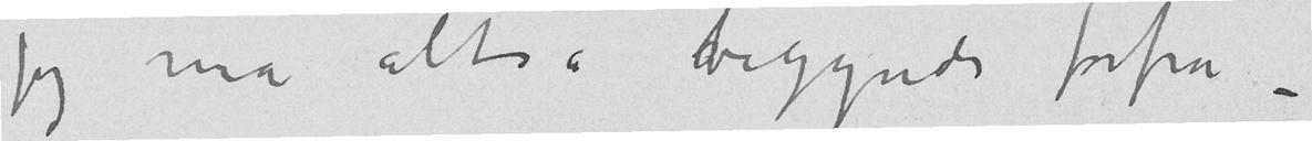Jeg må altså begynde forfra –
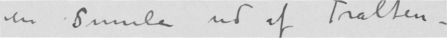en Smule ud af Tralten –
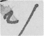2)
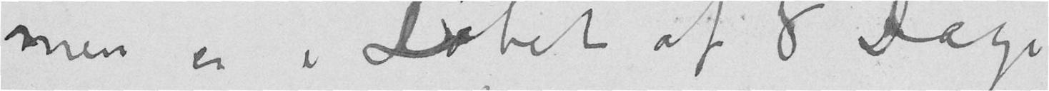men er i Løbet af 8 Dage
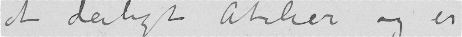et deiligt Atelier og er
